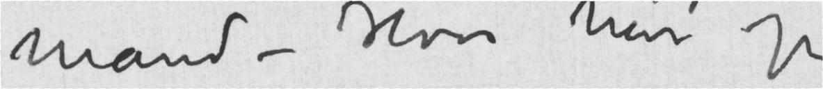mand – Hvor har jeg
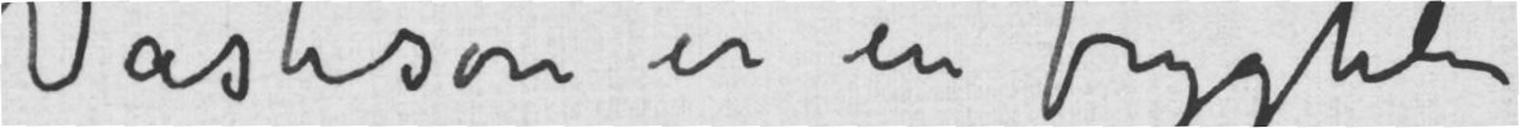Vasteson er en frygtet
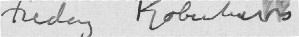Fredag Kjøbenhavn
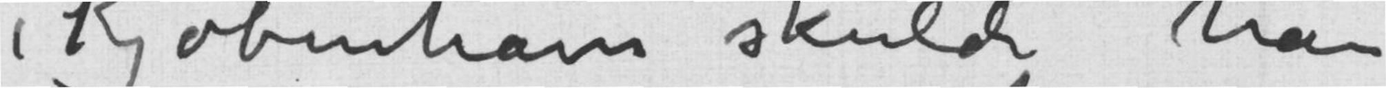i Kjøbenhavn skulde han
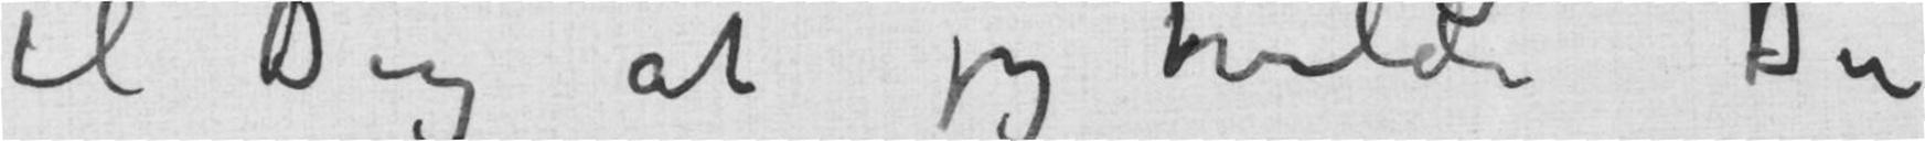til Dig at jeg vilde Du
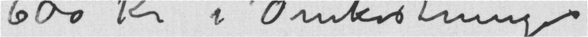600 Kr i Omkostninger
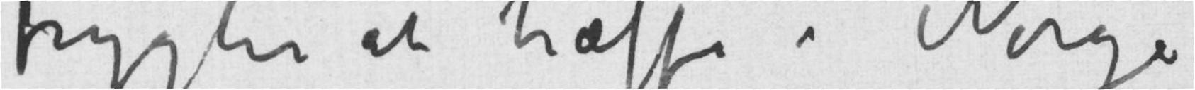frygter at træffe i Norge
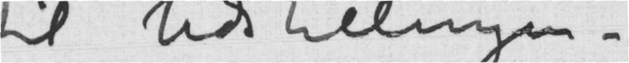til Udstillingen –
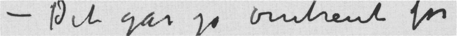Det går jo omtrent for
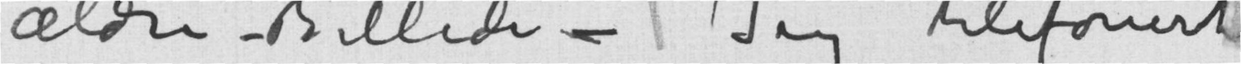ældre Billede – Jeg telefonerte
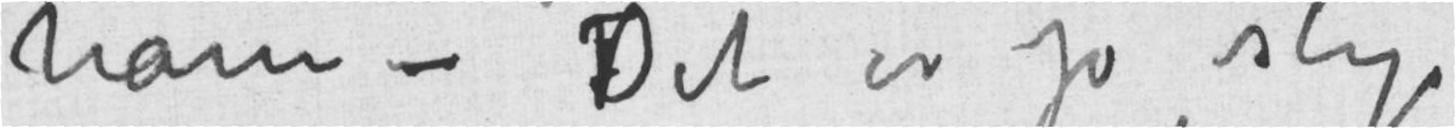ham – Det er jo slige
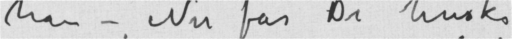han – «Nu får De huske
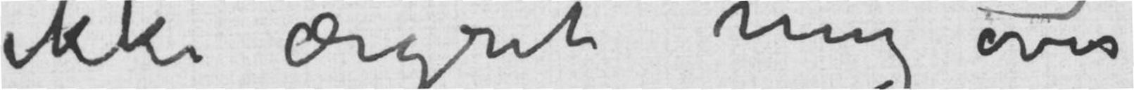ikke ærgret mig over
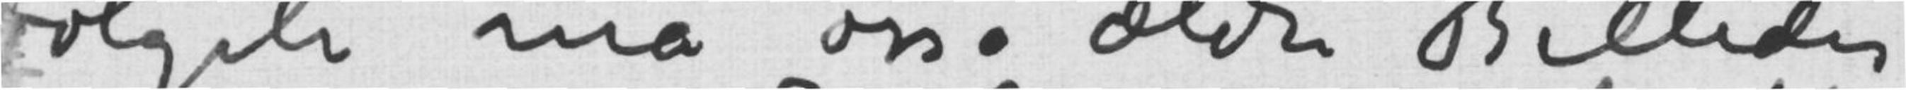følgeli må osså ældre Billeder
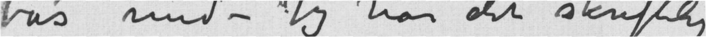tas med – Jeg har det skriftlig
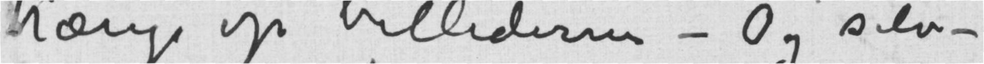hænge op billederne – Og selv-
